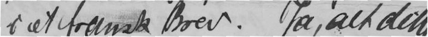i et fransk Brev. Ja, alt dette
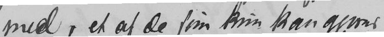med, et af De som kun kan gjøres
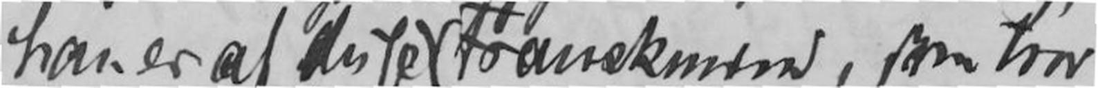han er af disse Franskmænd, som tror
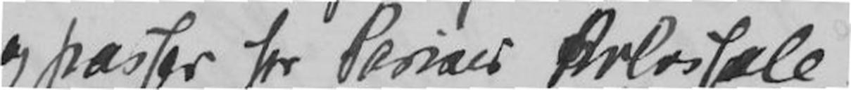og passer for Pariaer Arlossale
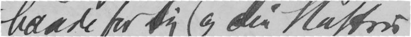baade for Dig og din Hustru
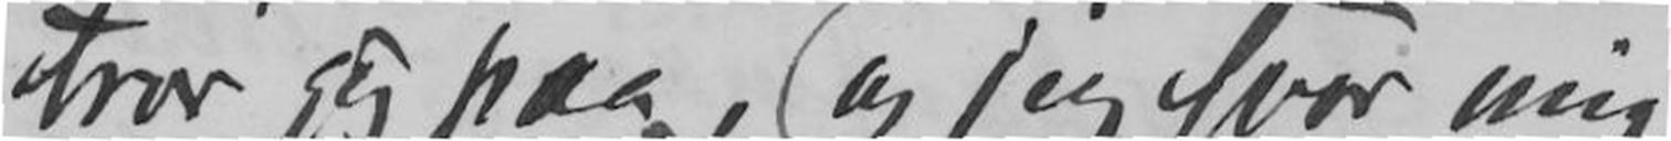tror jeg paa, og jeg svor mig
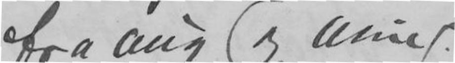fra mig og mine.
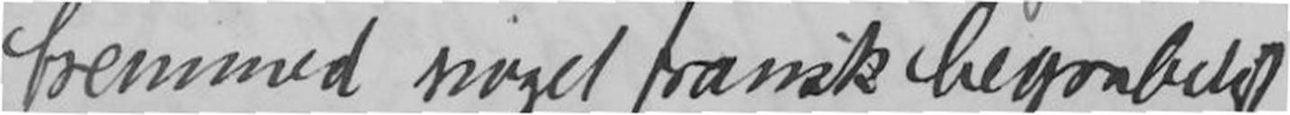fremmed noget fransk begribeligt
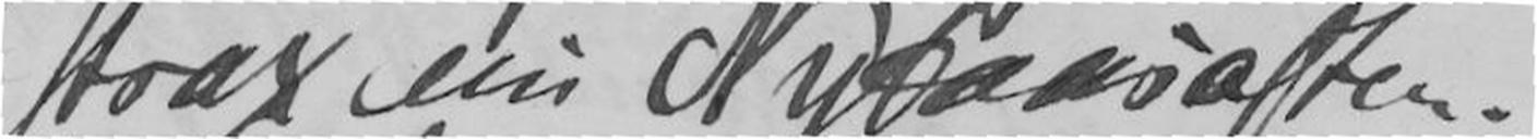strax nu Nytaarsaften.
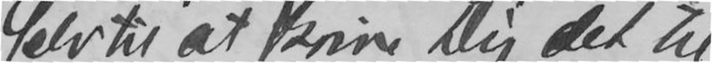selv til at skrive Dig det til
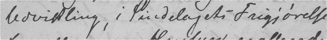Udvikling, i Sindelagets Frigjørelse
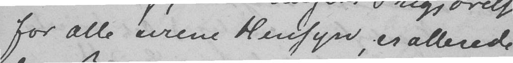for alle urene Hensyn, er allerede
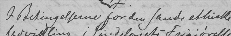I Betingelserne for den sande ethiske
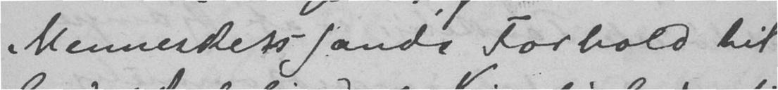Menneskets sande Forhold til
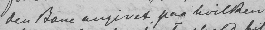den Bane angivet, paa hvilken
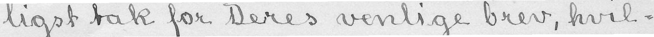ligst tak for Deres venlige brev, hvil-
